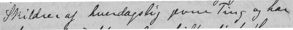Skildrer af hverdagslig jevne Ting og har
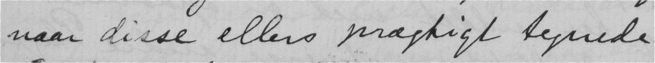naar disse ellers prægtigt tegnede
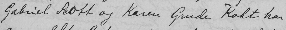Gabriel Scott og Karen Grude Koht har
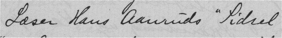Læser Hans Aanruds "Sidsel
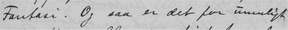Fantasi. Og saa er det for umuligt
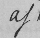af
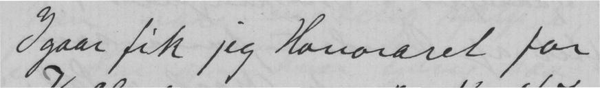Igaar fik jeg Honoraret for
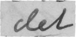det
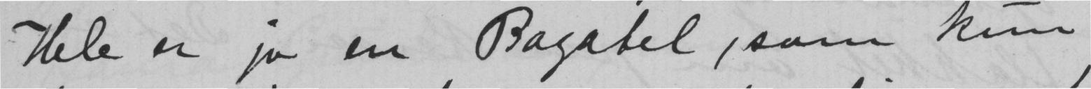Hele er jo en Bagatel, som kun
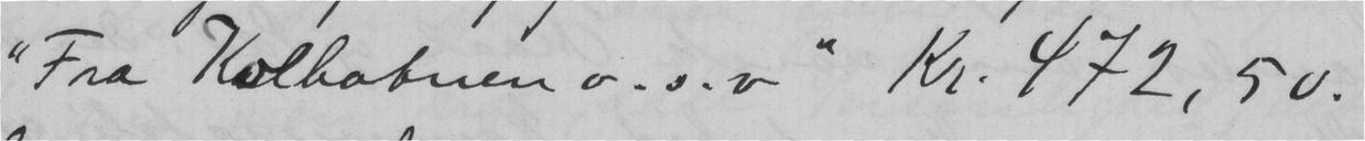"Fra Kolbotnen o.s.v" Kr. 472, 50.
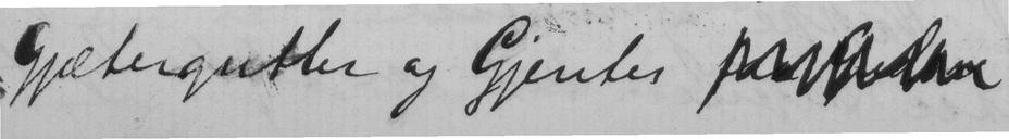Gjætergutter og Gjenter
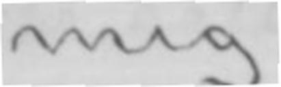mig
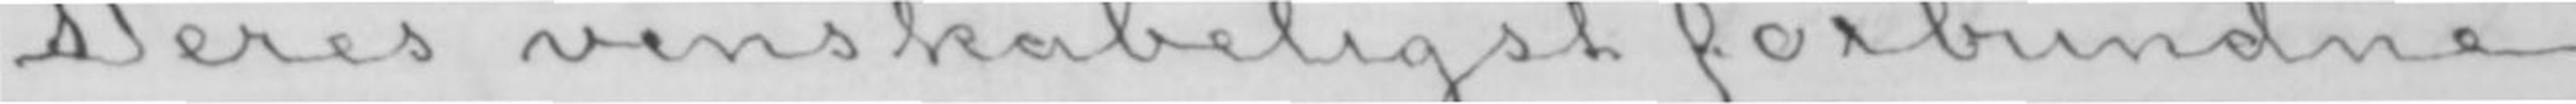Deres venskabeligst forbundne
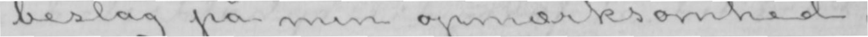beslag på min opmærksomhed,
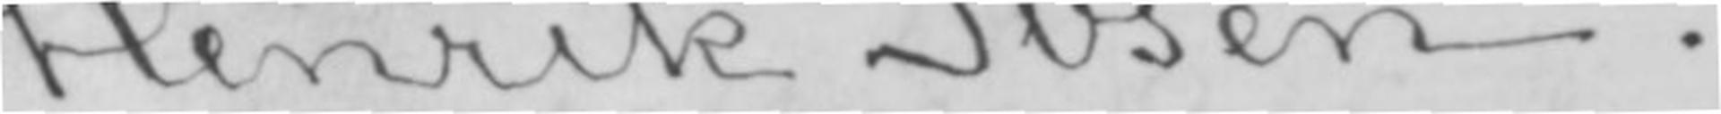Henrik Ibsen.
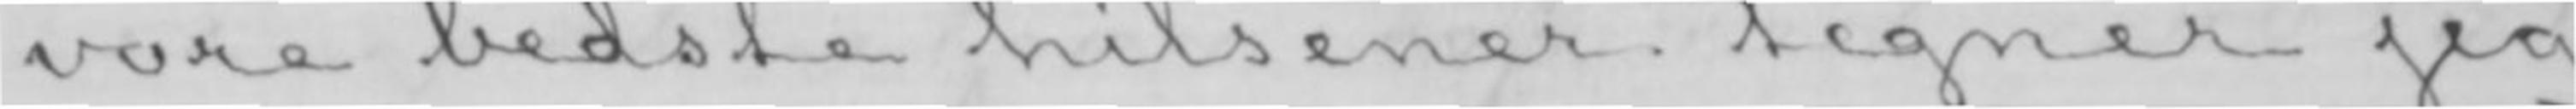vore bedste hilsener tegner jeg
